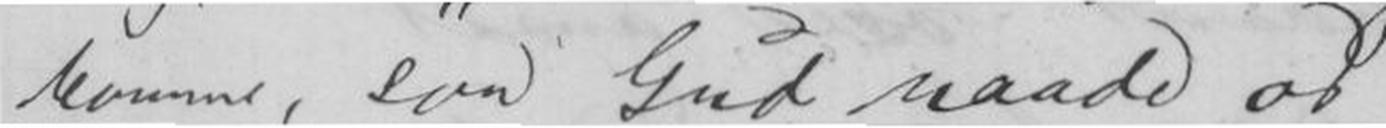komme, saa Gud naade os.
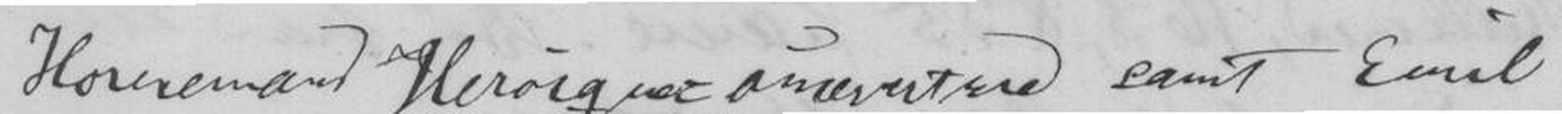Horneman Heroique=ouverture samt Emil
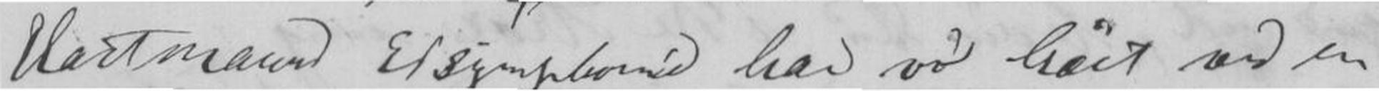Hartmans Essymphoni har vi hørt ved en
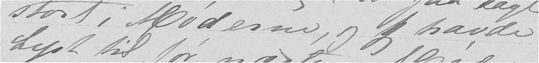stort i Møderne, og jeg havde
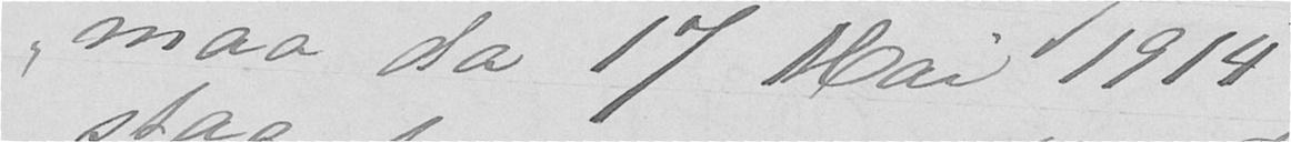maa da 17 Mai 1914
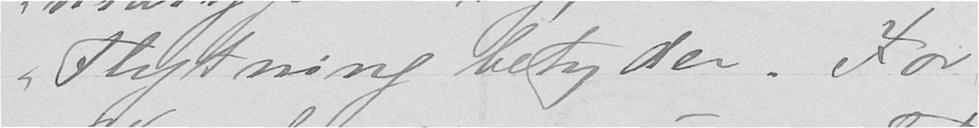Flytning betyder. For
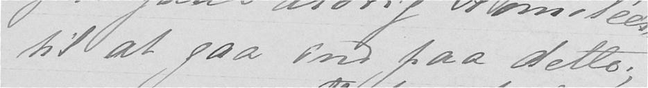til at gaa ind paa dette;
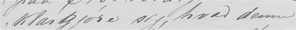klargjøre sig, hvad denne
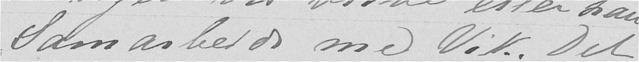Samarbeid med Vik. Det
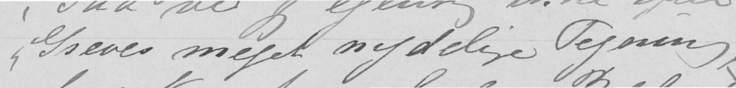Greves meget nydelige Tegning,
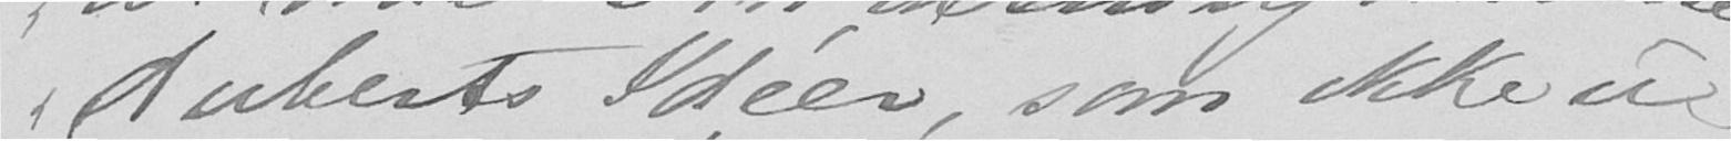Auberts Idéer, som ikke u-
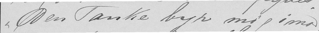Den Tanke byr mig imod,
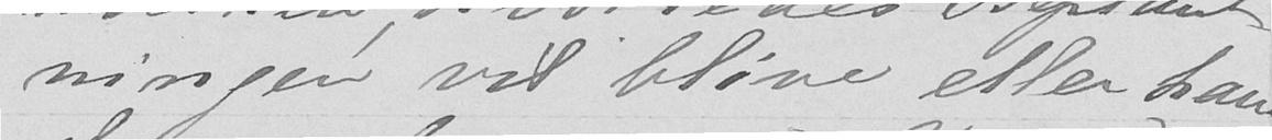ningen vil blive eller hans
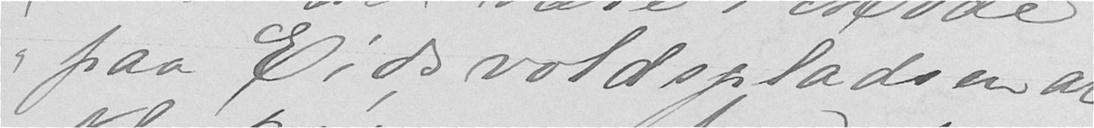paa Eidsvoldspladsen at
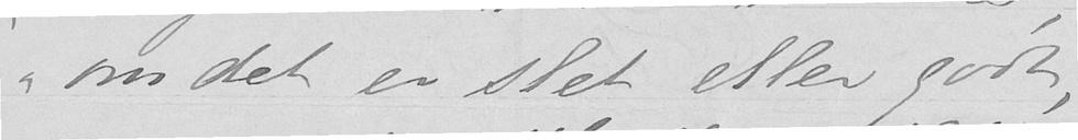om det er slet eller godt,
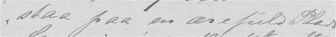staa paa en ærefuld Plads
